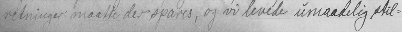retninger maatte der spares, og vi levede umaadelig stil-
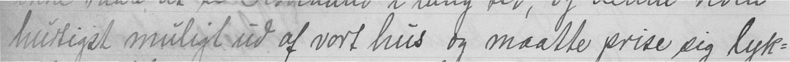hurtigst muligt ud af vort hus og maatte prise sig lyk-
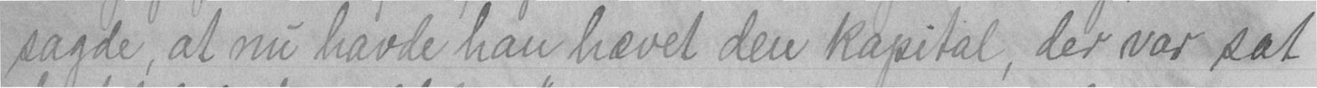sagde, at nu havde han hævet den kapital, der var sat
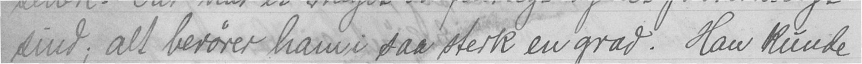sind; alt berører ham i saa sterk en grad. Han kunde
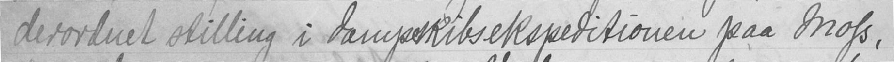derordnet stilling i dampskibsekspeditionen paa Mofs,
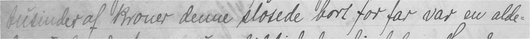tusinder af kroner denne sløsede bort for far var en alde-
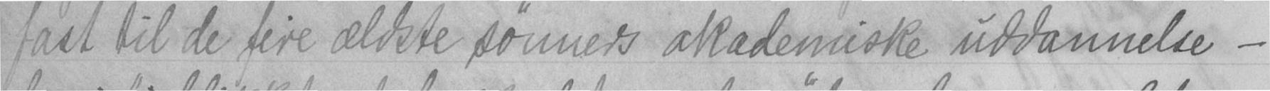fast til de fire ældste sønners akademiske uddannelse -
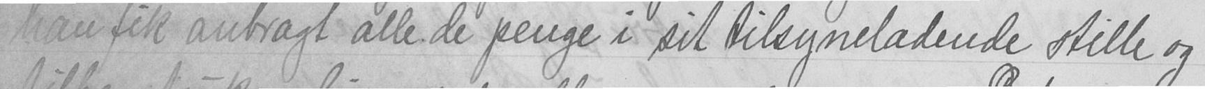han fik anbragt alle de penge i sit tilsyneladende stille og
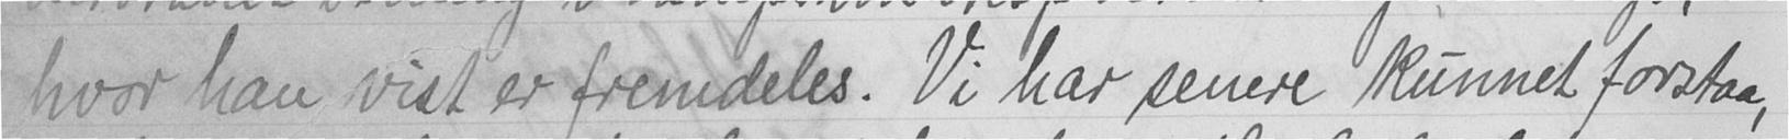hvor han vist er fremdeles. Vi har senere kunnet forstaa,
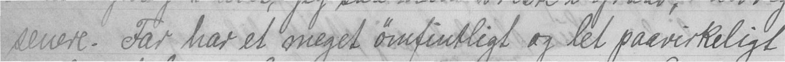senere. Far har et meget ømfintligt og let paavirkeligt
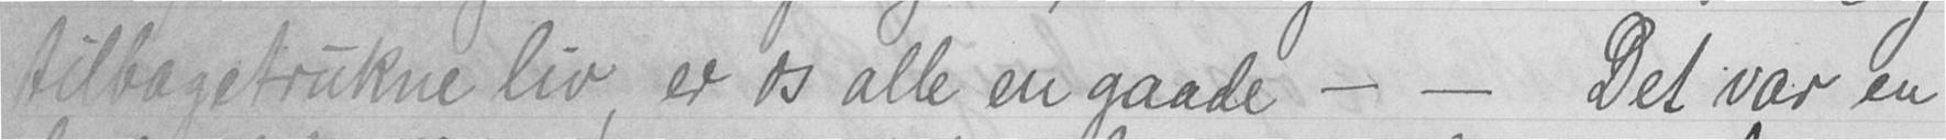tilbagstrukne liv, er os alle en gaade - - Det var en
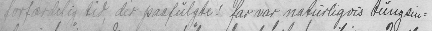forfærdelig tid, der paafulgte! far var naturligvis tungsin-
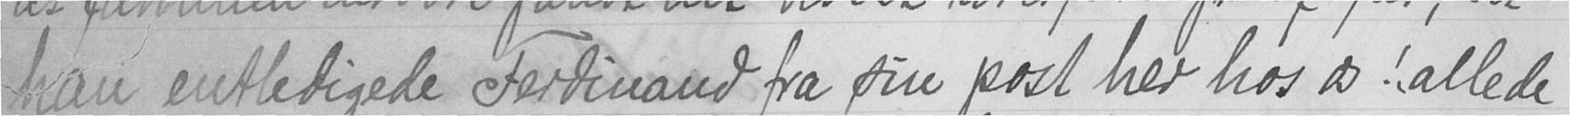han entledigede Ferdinand fra sin post her hos os! allede
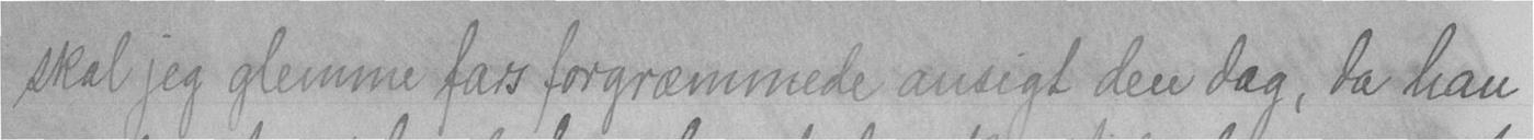skal jeg glemme fars forgræmmede ansigt den dag, da han
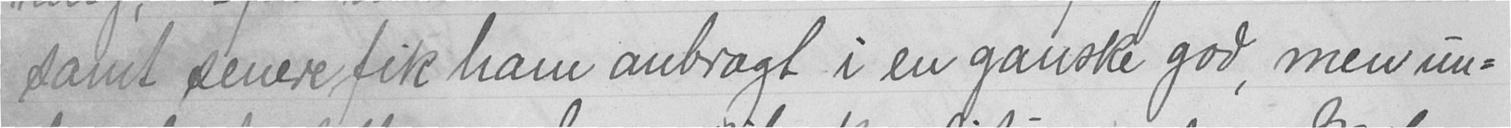samt senere fik ham anbragt i en ganske god, men un-
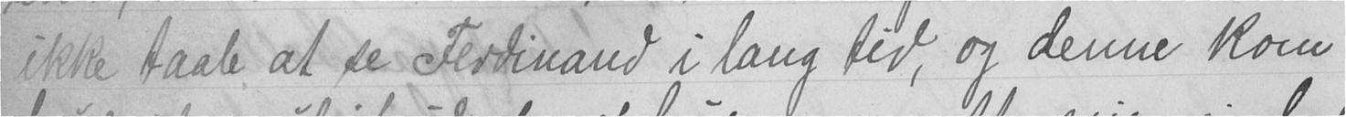ikke taale at se Ferdinand i lang tid, og denne kom
